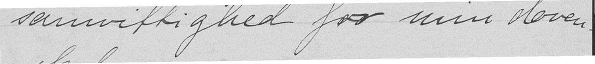samvittighed fra min doven-
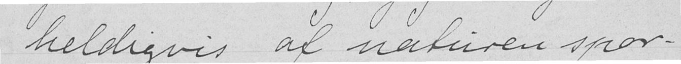heldigvis af naturen spor-
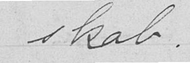skab.
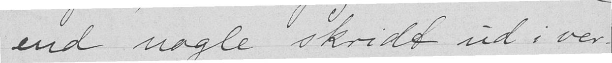end nogle skridt ud i ver-
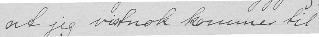at jeg visnok kommer til
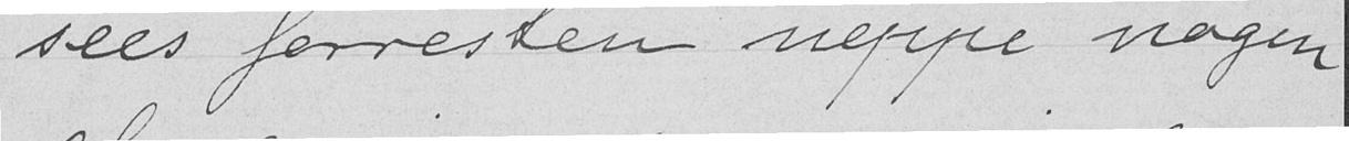sees forresten neppe nogen
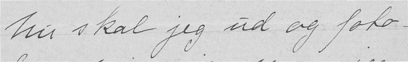Nu skal jeg ud og foto-
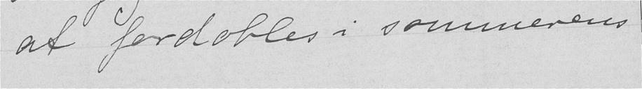at fordøbles i sommerens
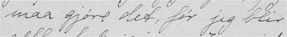maa gjøre det, før jeg blir
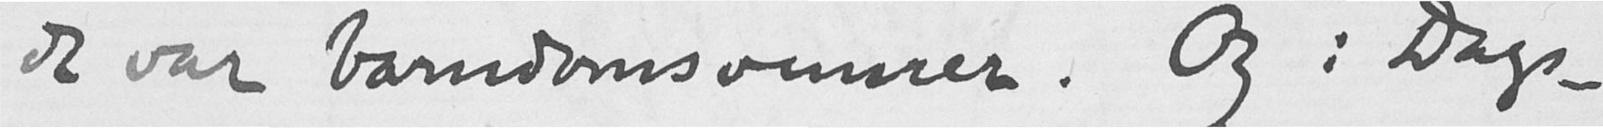De var barndomsvenner. Og i "Dags-
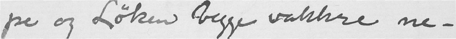pe og Løken begge vakkre ne-
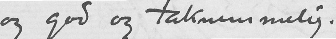og god og taknemmelig.
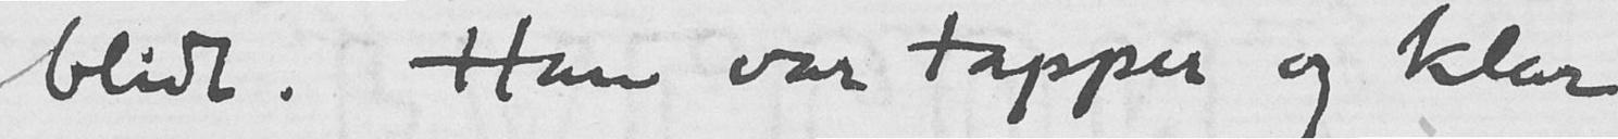blidt. Han var tapper og klar
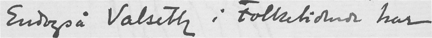Endogså Valseth i "Folketidende" har
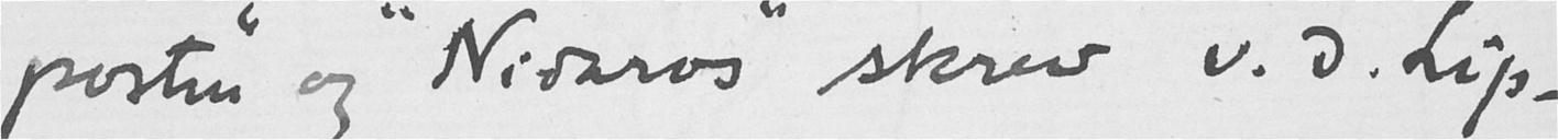posten" og "Nidaros" skrev v. d. Lip-
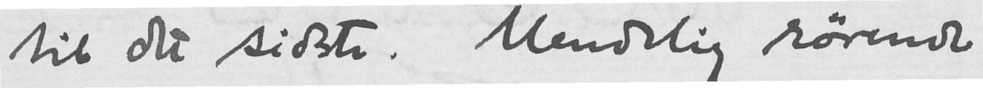til det sidste. Uendelig rørende
